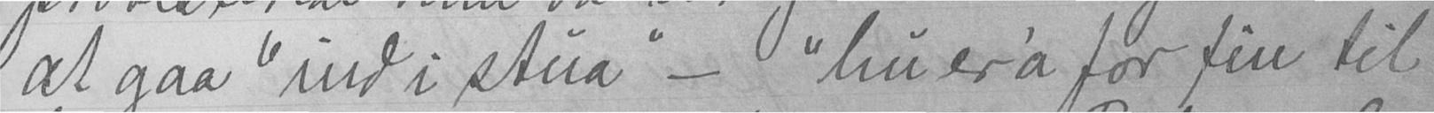at gaa "ind i stua" - "hu er 'a for fin til
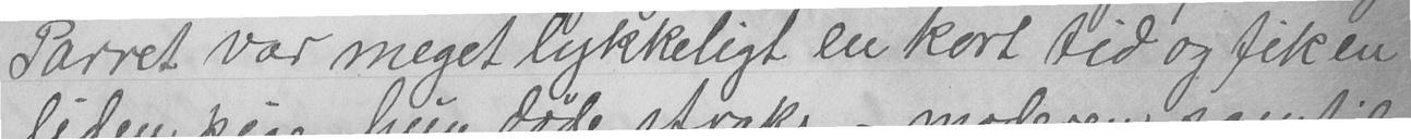Parret var meget lykkeligt en kort tid og fik en
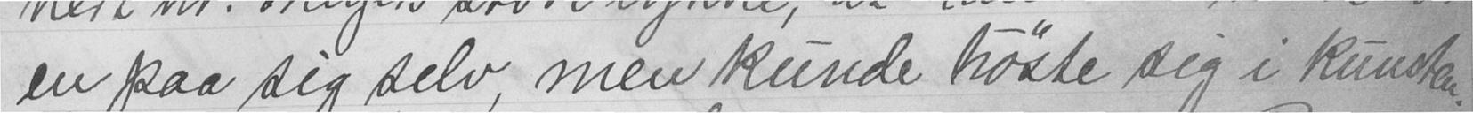en paa sig selv, men kunde trøste sig i kunsten.
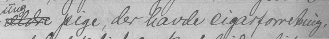ældre pige, der havde cigarforretning.
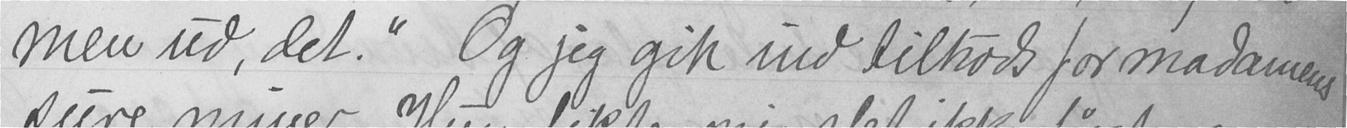men ud, det." Og jeg gik ind tiltrods for madamens
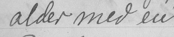alder med en
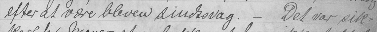efter at være bleven sindssvag. - Det var sik-
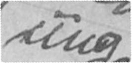ung
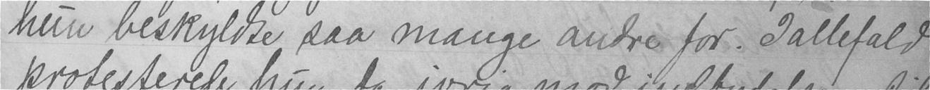hun beskyldte saa mange andre for. Iallefald
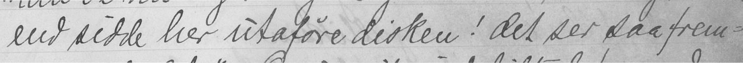end sidde her utaføre disken! det ser saa frem-
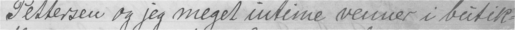Pettersen og jeg meget intime venner i butik-
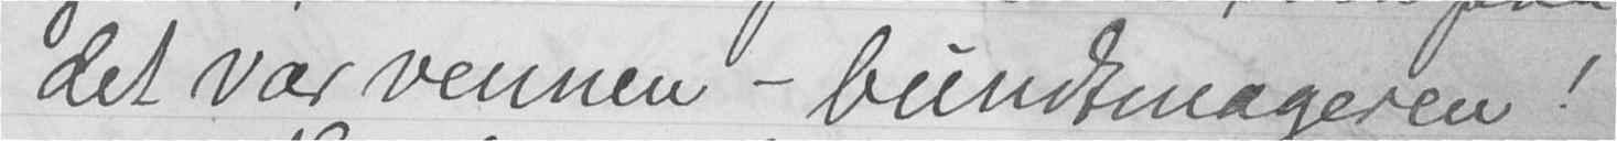det var vennen - bundtmageren!
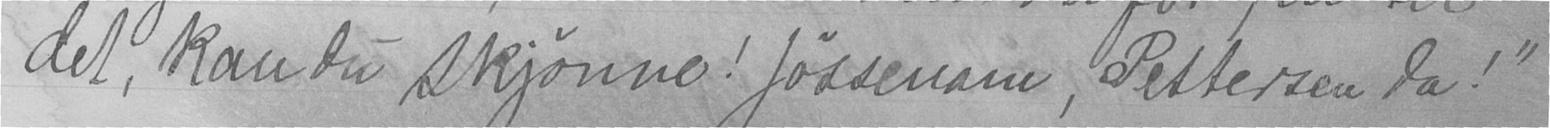det, kan du skjønne! Jøssenam, Pettersen da!"
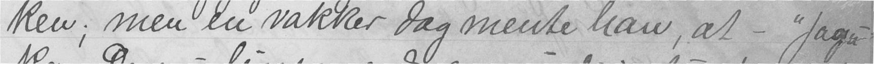ken; men en vakker dag mente han, at - "Jagu
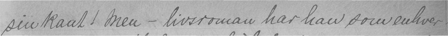sin kant! men - livsroman har han som enhver
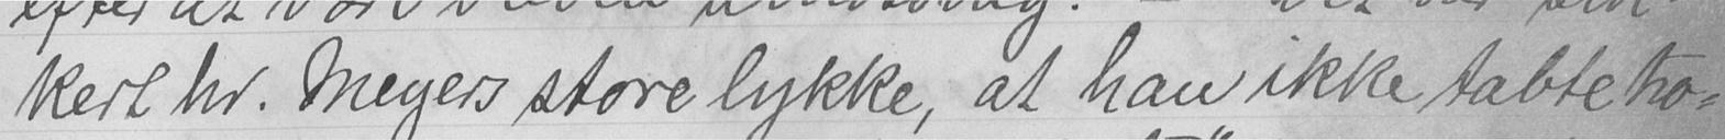kert hr. Meyers store lykke, at han ikke tabte tro-
